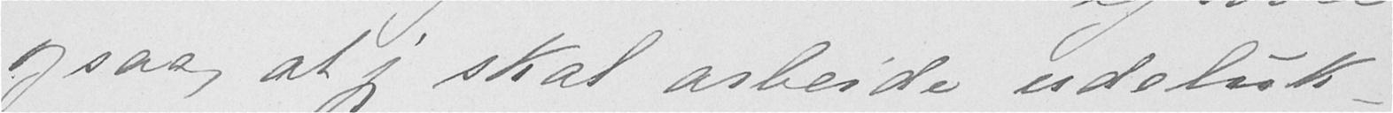ogsaa, at jeg skal arbeide udeluk-
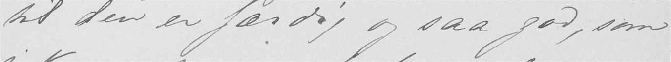til den er færdig og saa god, som
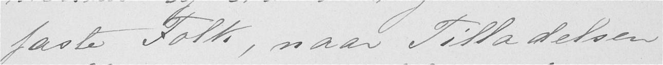faste Folk, naar Tilladelsen
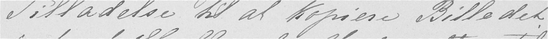Tilladelse til at kopiere Billedet
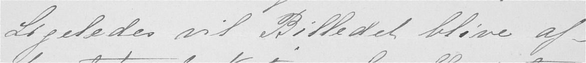Ligeledes vil Billedet blive af-
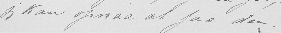jeg kan opnaa at faa den.
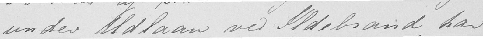under Udlaan ved Ildebrand har
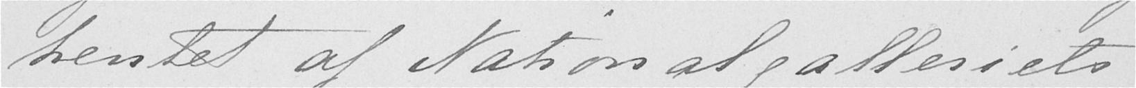hentet af Nationalgalleriets
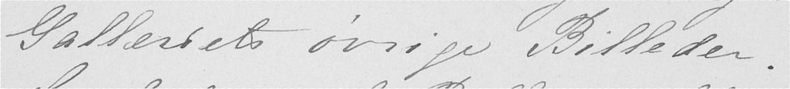Galleriets øvige Billeder.
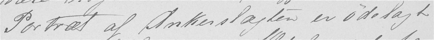Portræt af Ankerslægten er ødelagt
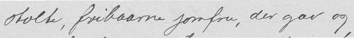stolte, fribaarne jomfru, der gav og
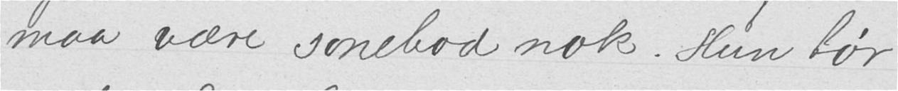maa være sonebod nok. Hun bør
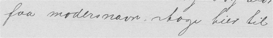faa modersnavn. Aage tier til
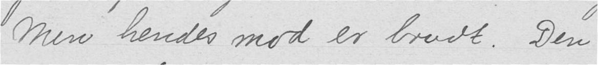Men hendes mod er brudt. Den
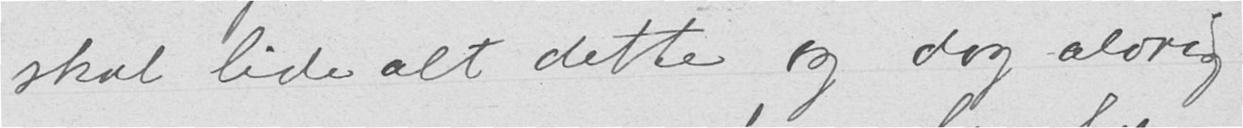skal lide alt dette og dog aldrig
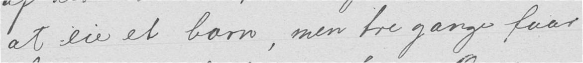at eie et barn, men tre ganger faar
